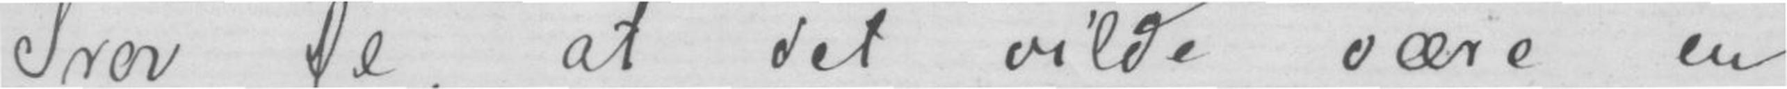Tror De, at det vilde være en
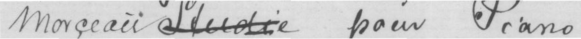Morceau Studie pour Piano
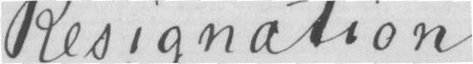Resignation.
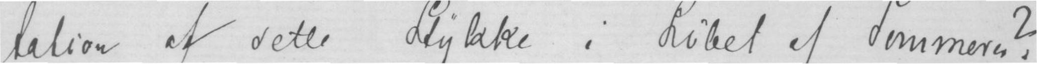talion af dette Stykke i Løbet af Sommeren?
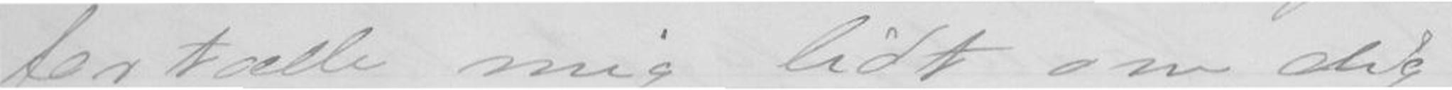fortælle mig lidt om dig
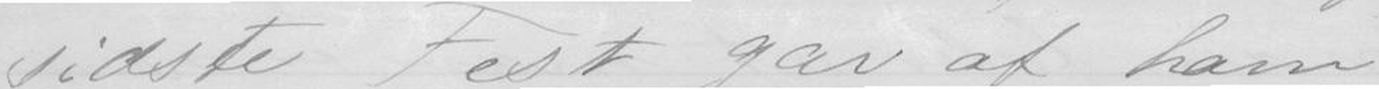sidste Fest gav af ham
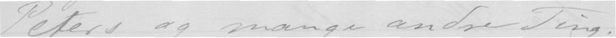Peters og mange andre Ting.
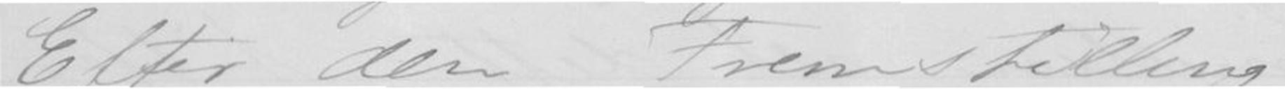Efter den Fremstilling
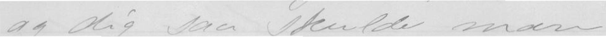og dig saa skulde man
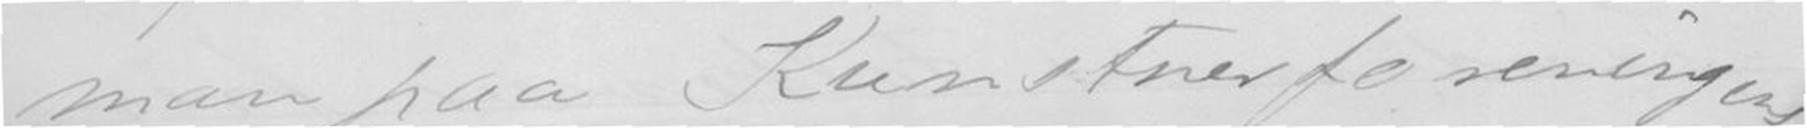man paa Kunstnerforeningens
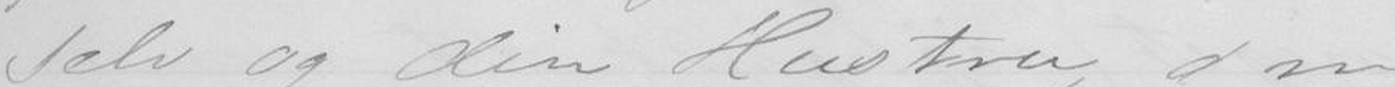selv og din Hustru, om
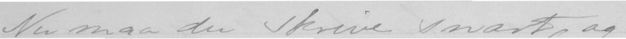Nu maa du skrive snart, og
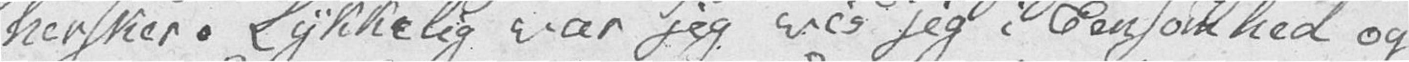hersker. Lykkelig var jeg vis jeg i Eensomhed og
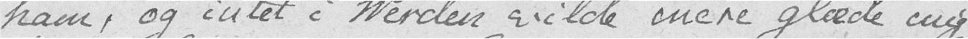ham, og intet i Verden vilde mere glæde mig
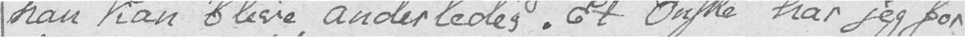han kan blive anderledes. Et Ønske har jeg for
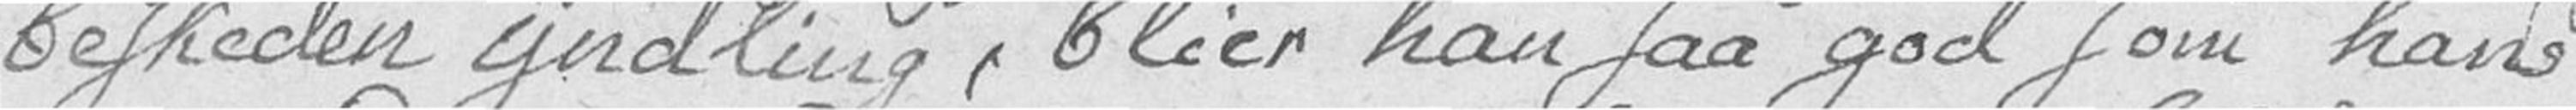beskeden Yndling, blier han saa god som hans
